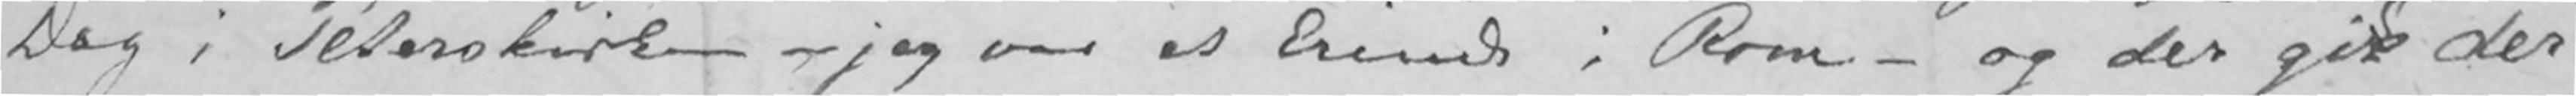Dag i Peterskirken- jeg var et Erinde i Rom- og der gikgi der
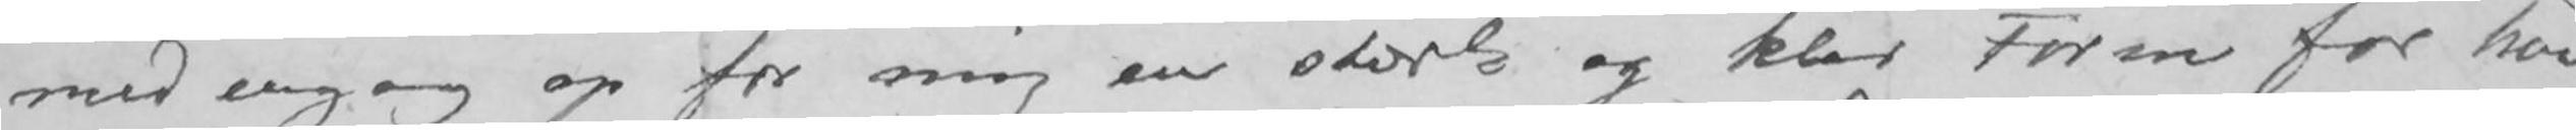med engang op for mig en stærk og klar Form for hvad
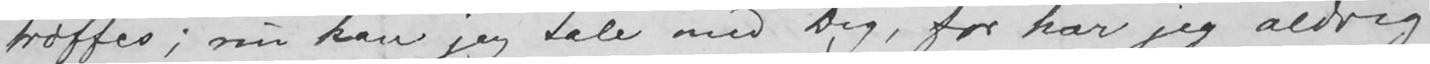træffes; nu kan jeg tale med Dig, før har jeg aldrig
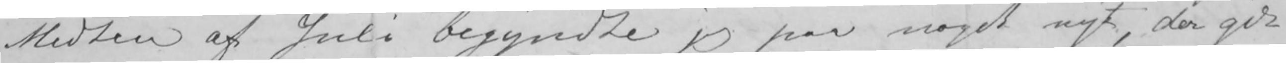Midten af Juli begyndte jeg paa noget nyt, der gik
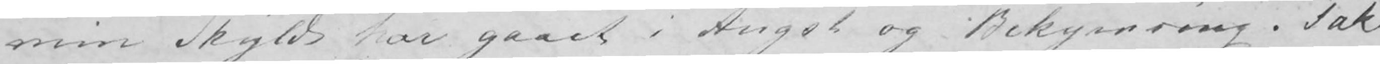min Skyld har gaaet i Angst og Bekymring. Tak
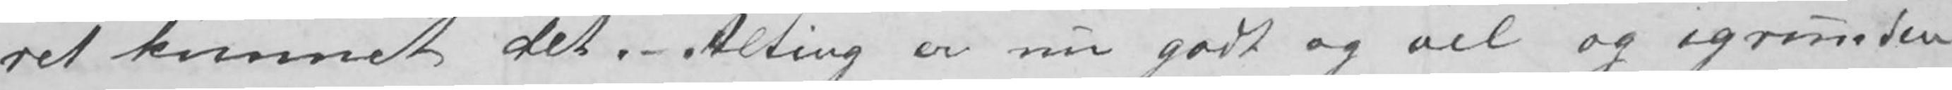ret kunnet det.- Alting er nu godt og vel og igrunden
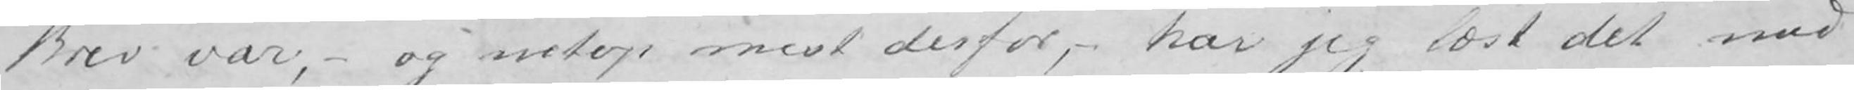Brev var,- og netop mest derfor,- har jeg læst det med
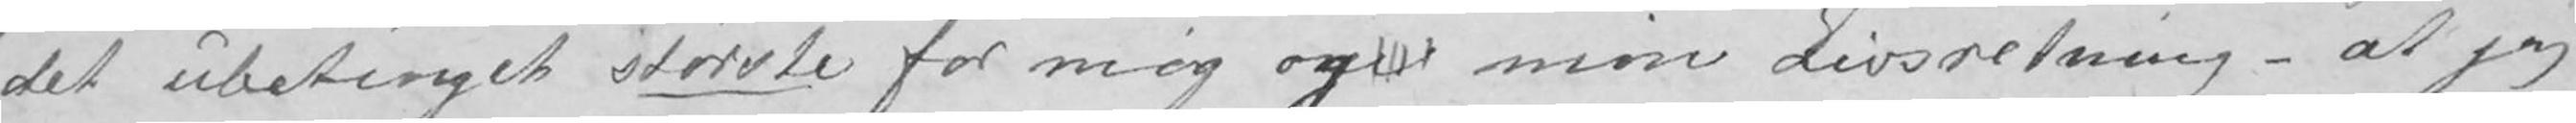det ubetinget største for mig ogo min Livsretning- at jeg
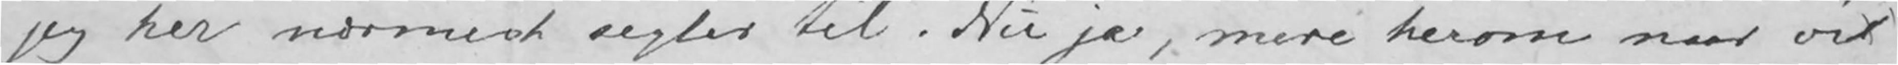jeg her nærmest sigter til. Nu ja, mere herom naar vi
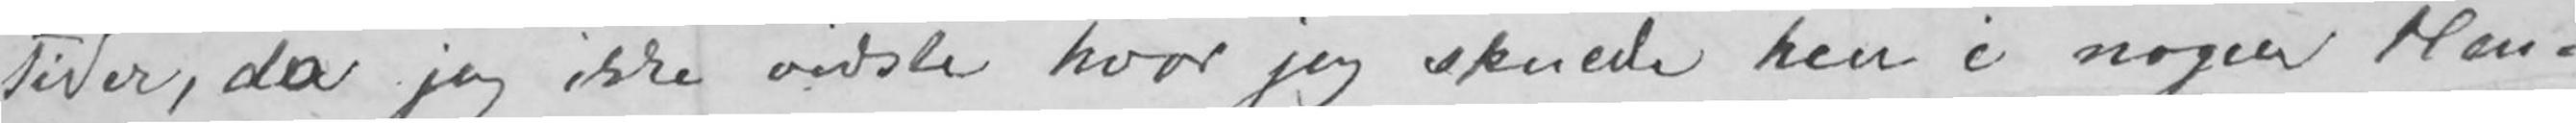Tider, da jeg ikke vidste hvor jeg skulde hen i nogen Hen-
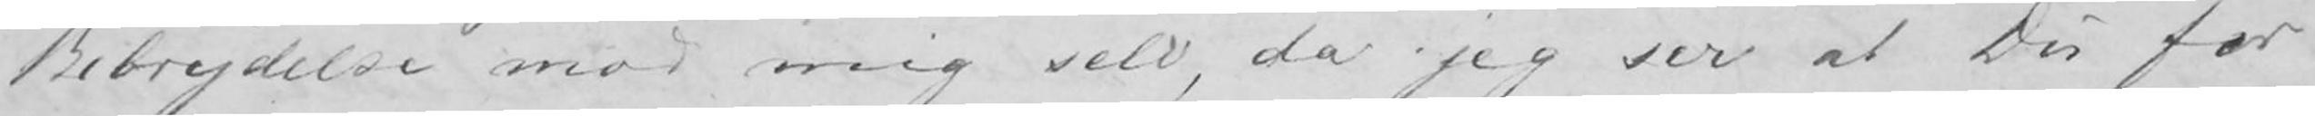Bebrejdelse mod mig selv, da jeg ser at Du for
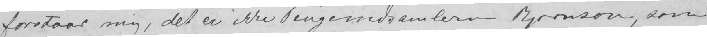forstaar mig, det er ikke Pengeindsamleren Bjørnson, som
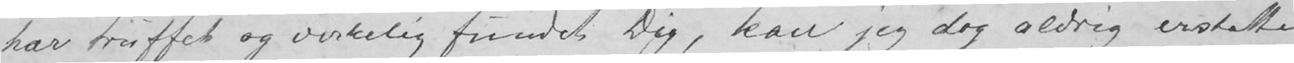har truffet og virkelig fundet Dig, kan jeg dog aldrig erstatte
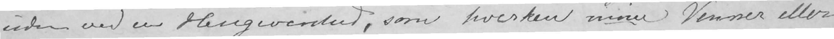uden ved en Hengivenhed, som hverken mine Venner eller
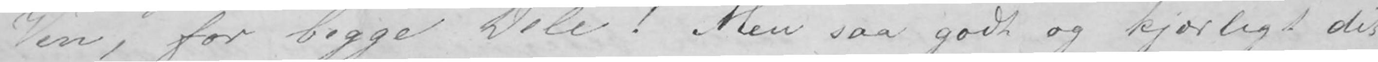Ven, for begge Dele! Men saa godt og kjærligt dit
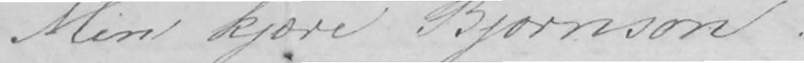Min kjære Bjørnson!
